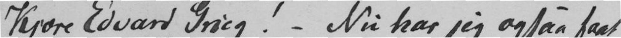Kjære Edvard Grieg! - Nu har jeg ogsaa faat
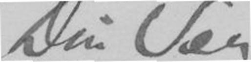Din Ven
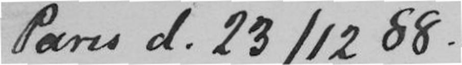Paris d. 23/12 88.
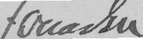Jonas Lie
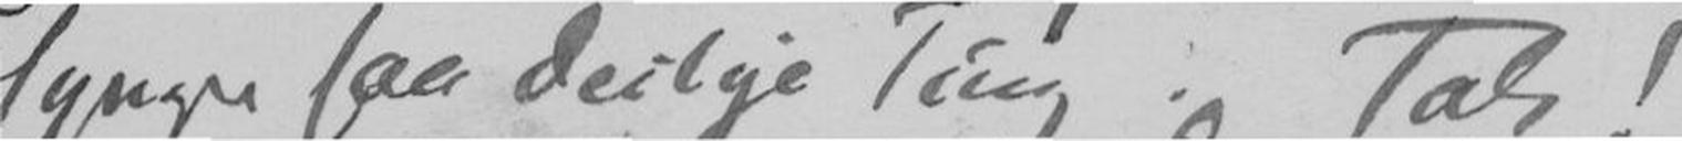synger saa deilige Ting. Tak!
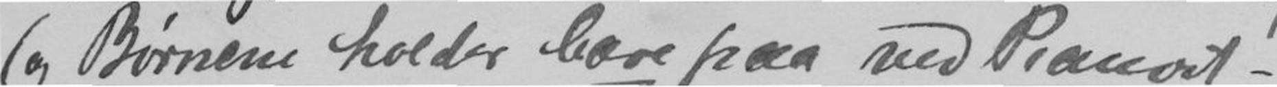og Børnene holder Bare paa ved Pianoet -
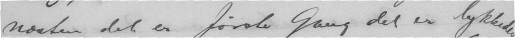næsten det er første Gang det er Lykkedes
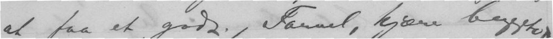at faa et godt. Farvel, kjære beggeto,
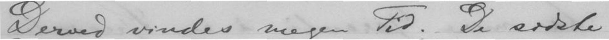Derved vindes megen Tid. De sidste
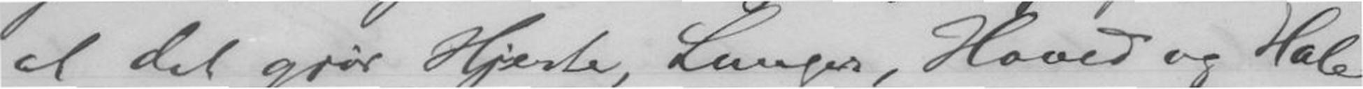at det gjør Hjerte, Lunger, Hoved og Hale
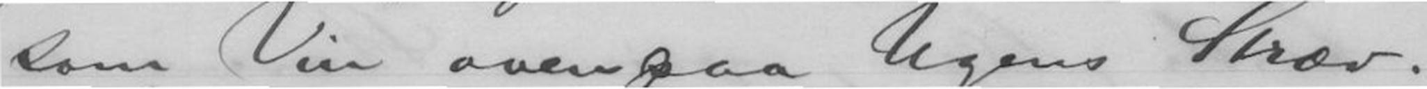som Vin ovenpaa Ugens Stæv.
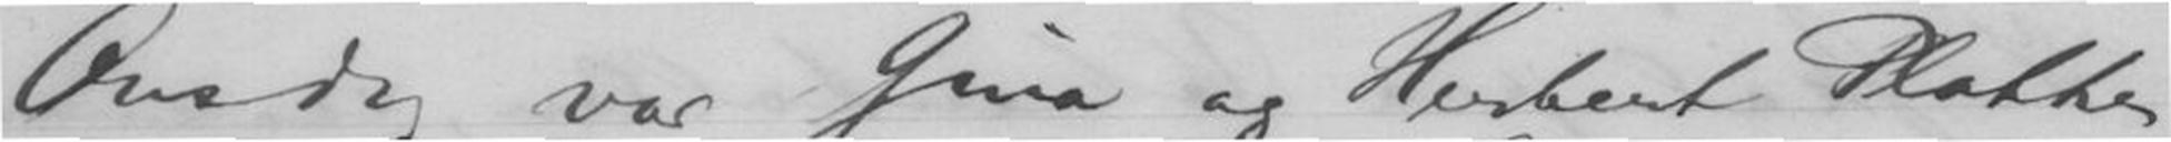Onsdag var Gina og Herbert Plathe
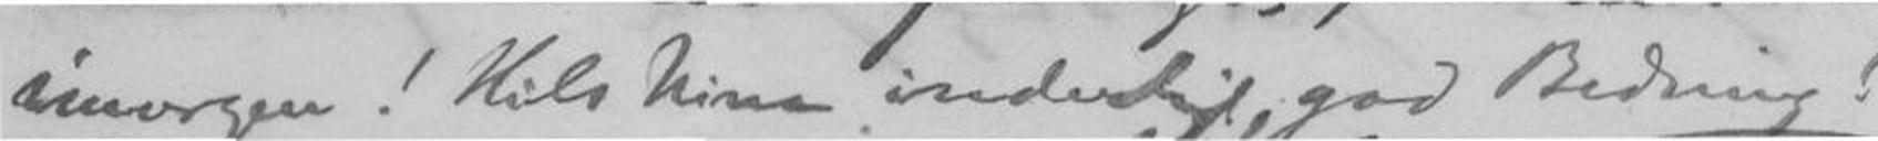imorgen! Hils Nina inderligt, god Bedring!
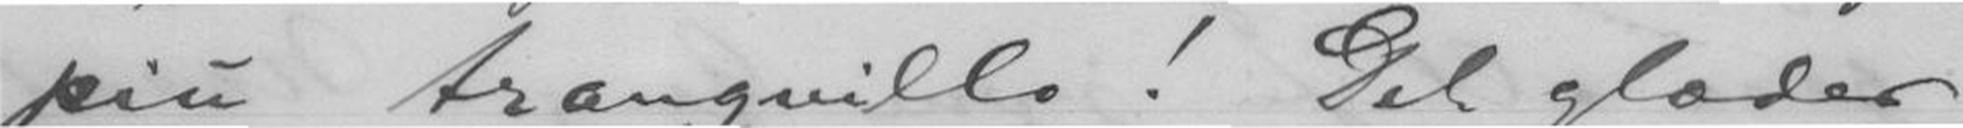piu tranqvillo! Det glæder
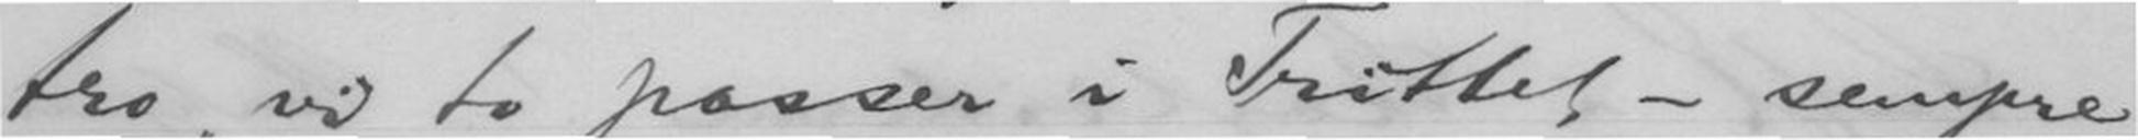tro, vi to passer i Trittet - sempre
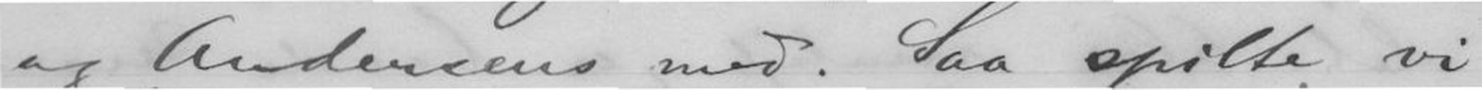og Andersens med. Saa spilte vi
